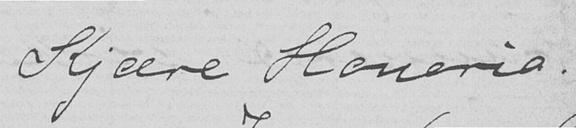Kjære Honoria!
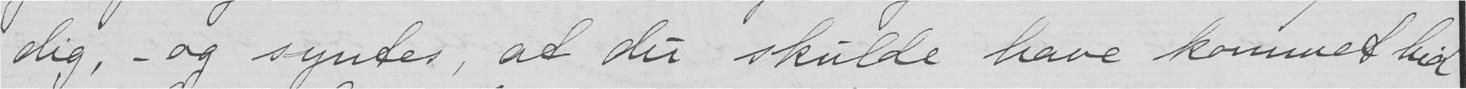dig, – og syntes, at du skulde have kommet hid
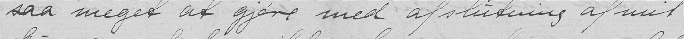saa meget at gjøre med afslutning af mit
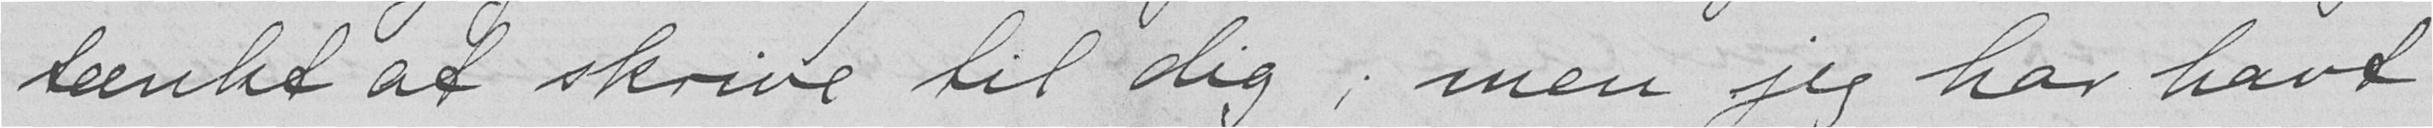tænkt at skrive til dig; men jeg har havt
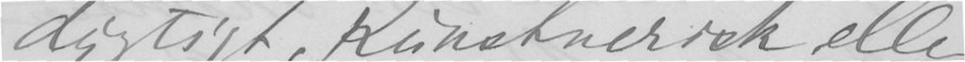dygtigt, kunstnerisk eller
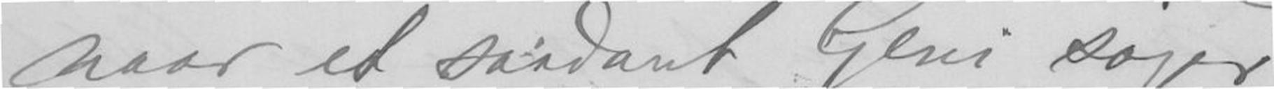naar et saadant Geni søger
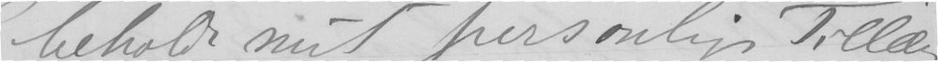beholde mit personlige Tillæg
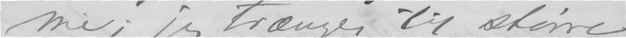me; jeg trænge til større
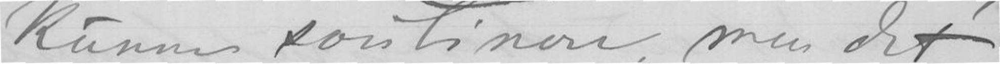kunne soritinon, men det
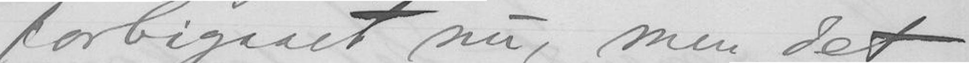forbigaaet nu, men det
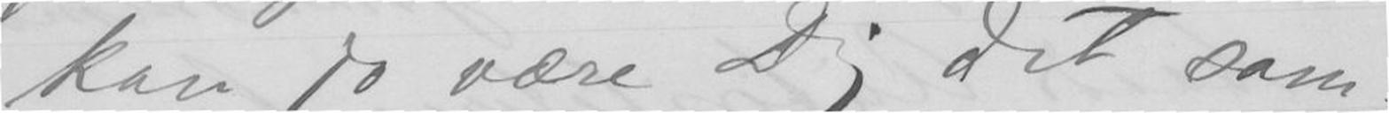kan jo være Dig det sam-
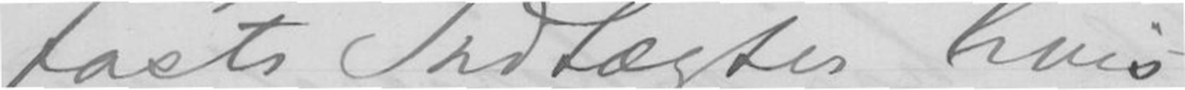faste Indtægter hvis
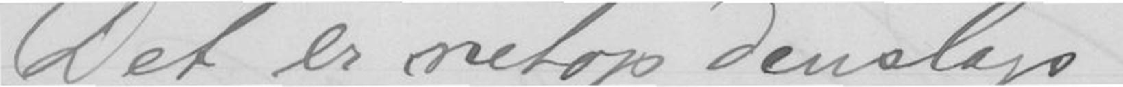Det er netop denslags
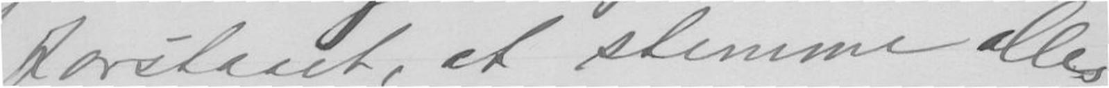forstaaet, at stemme alles
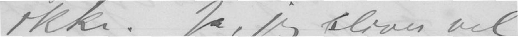ikke. Ja, jeg bliver vel
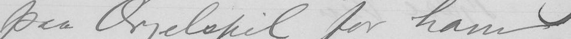paa Orgelspil for ham.
